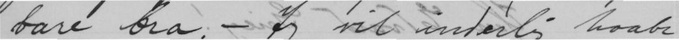bare bra. - Jeg vil inderlig haabe
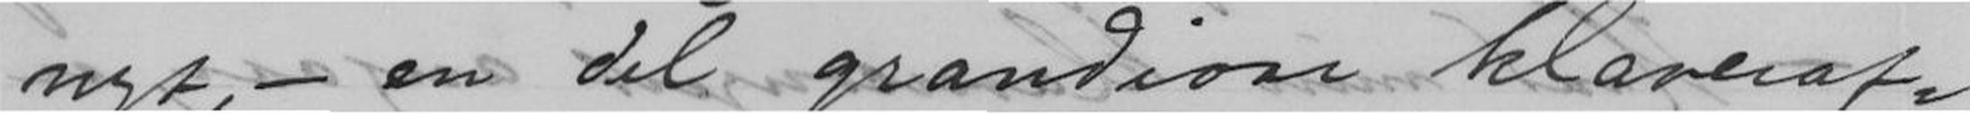nyt, - en del grandiose klaveraf
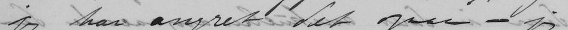har angret det ogsaa. - jeg
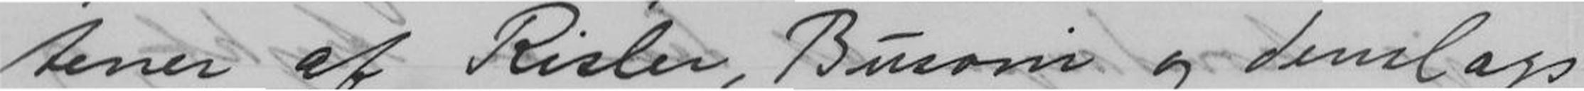tener af Risler, Busoni og denslags
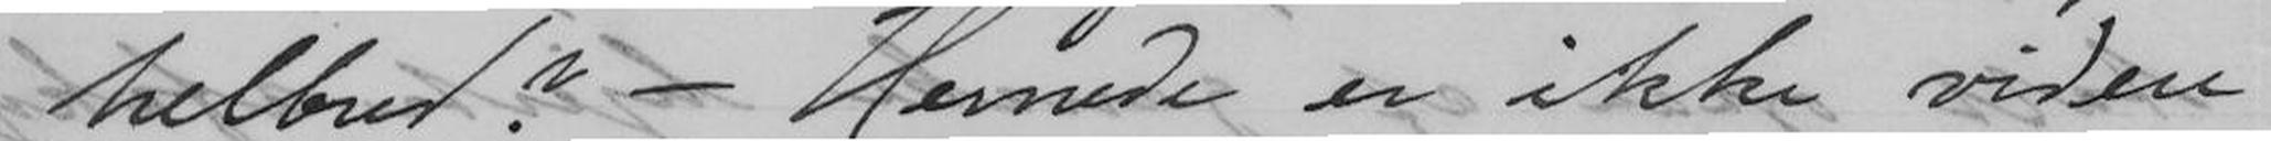helbred? - Hernede er ikke videre
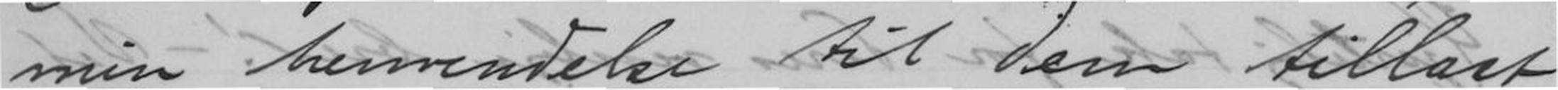min hendvendelse til dem tillast,
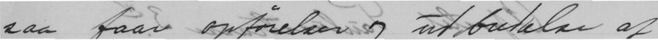saa faar opførelser og udbredelse af
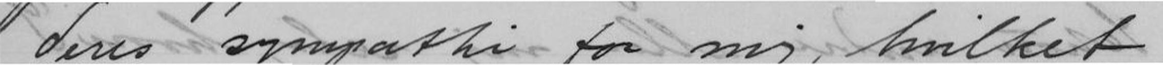deres sympathi for mig, hvilket
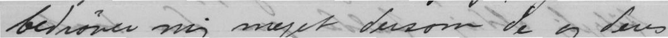bedrøver mig meget dersom de og
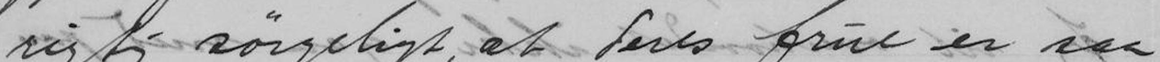rigtig sørgeligt, at deres frue er saa
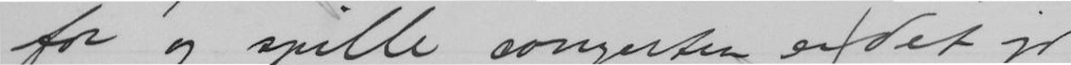for og spille conzerten er det jo
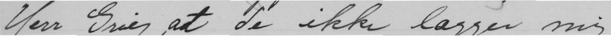Herr Grieg, at de ikke lægger mig
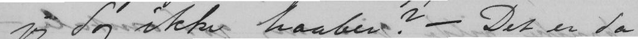jeg dog ikke haaber? - Det er da
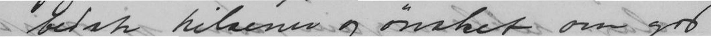bedste hilsener og ønsket om god
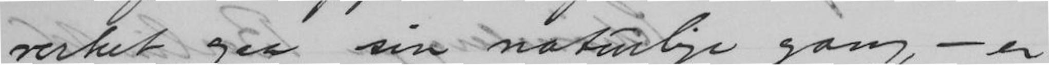verket gaa sin naturlige gang, - er
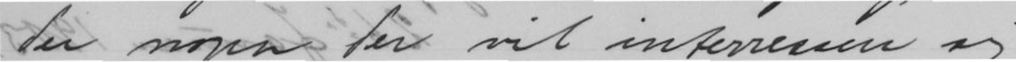der nogen der vil interressere sig
